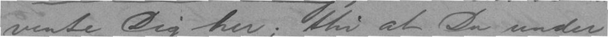vente Dig her; thi at Du under
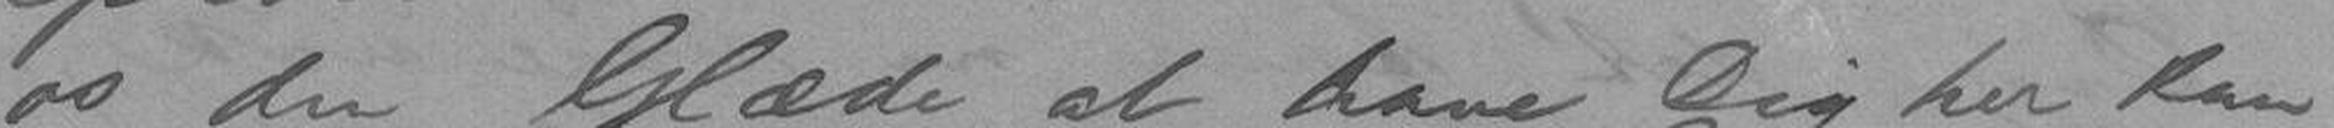os den Glæde at have Dig her, kan
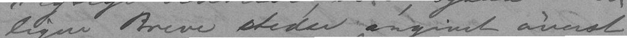ligere Breve stedse angivet øverst
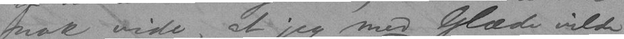nok vide, at jeg med Glæde vilde
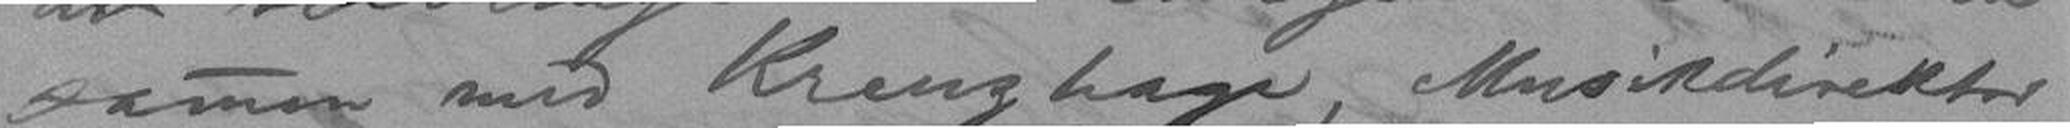sammen med Kreuzhage, Musikdirektør
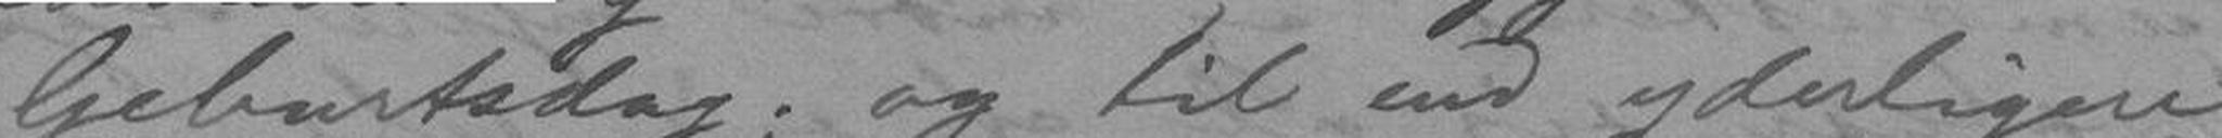Geburtsdag; og til end yderligere
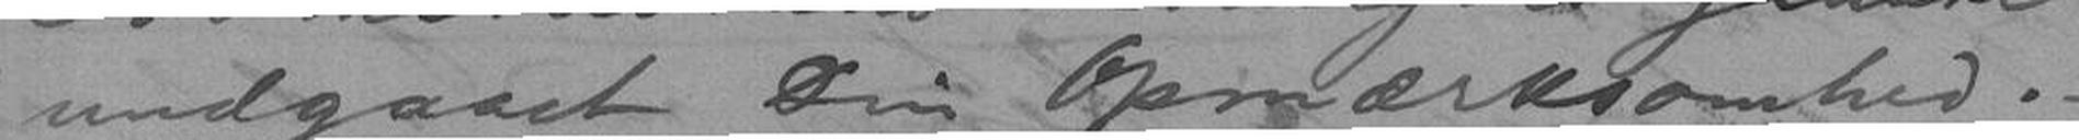undgaaet Din Opmærksomhed. -
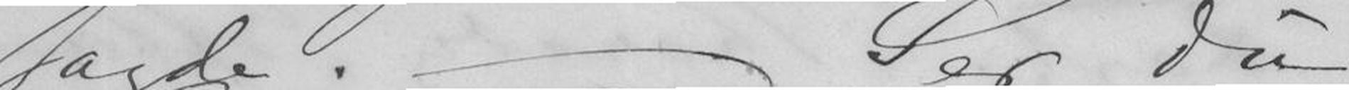sagde. ----- Ser du
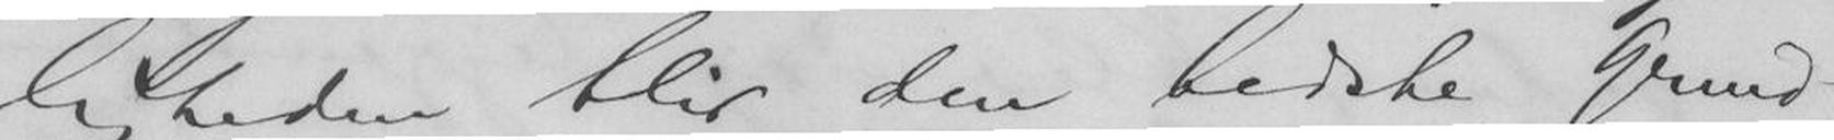ligheden blir den bedste Grund-
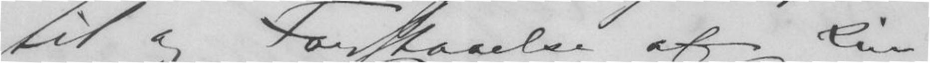til og Forstaaelse af sin
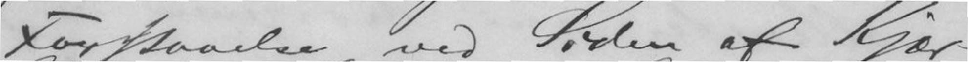Forstaaelse ved Siden af Kjær-
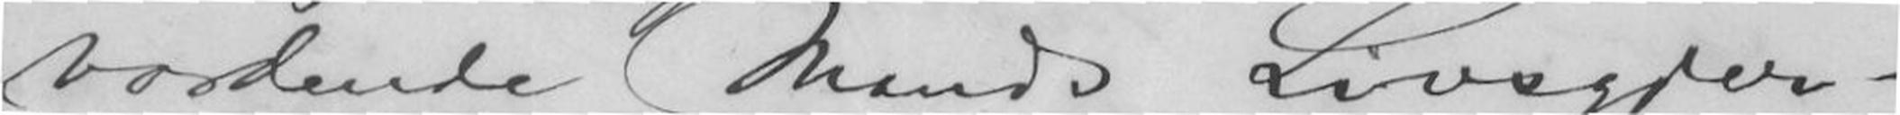vordende Mands Livsgjer-
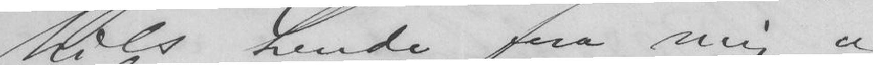hils hende fra mig og
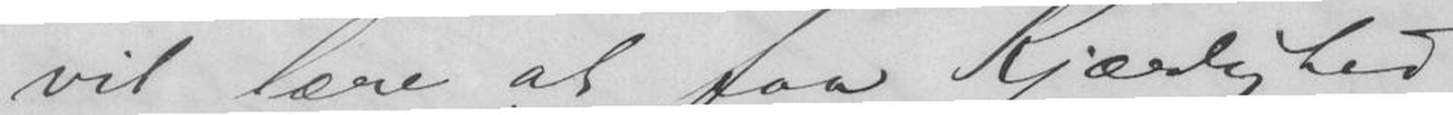vil lære at faa Kjærlighed
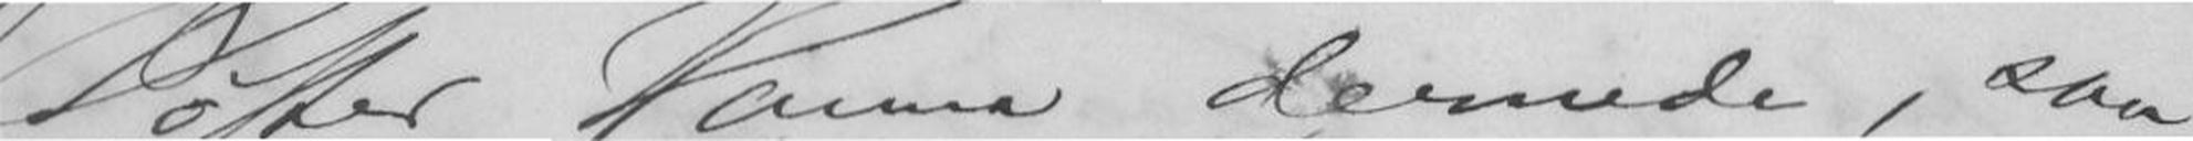Søster Hanna dernede, saa
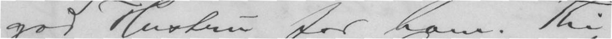god Hustru for ham. Thi
